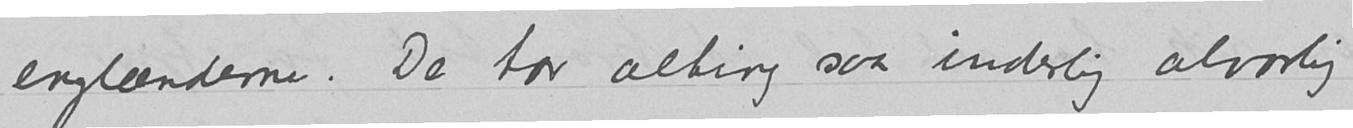englænderne. De tar alting saa inderlig alvorlig
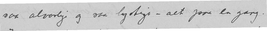saa alvorlige og saa lystige – alt paa en gang.
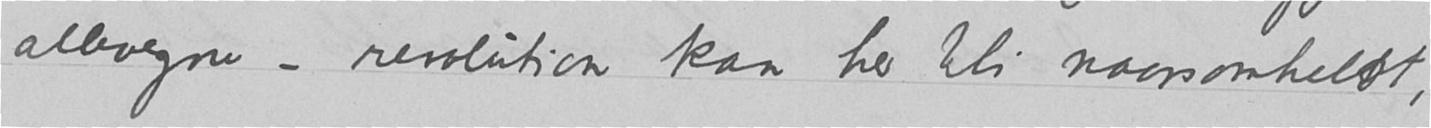allevegne – revolution kan her bli naarsomhelst,
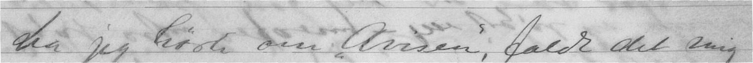da jeg hørte om Avisen, faldt det mig
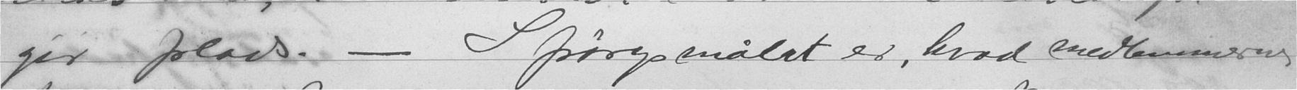gir plads. - I spørgsmålet er, hvad med Maasensnes
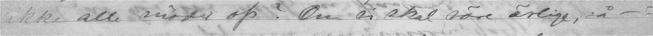ikke alle møder op? Om vi skal være ærlige, så -
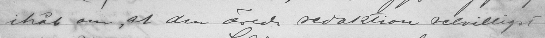i håb om, at den ærede redaktion velvilligst
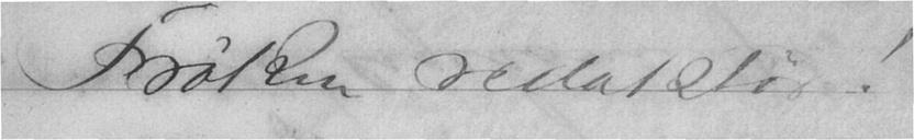Frøken redaktør!
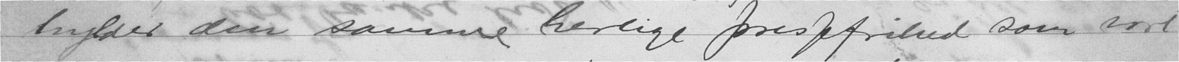hylder dem sammee herlige Jens Jefried som vort
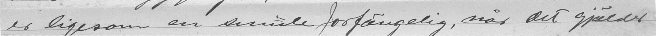er ligesom en smule forfengelig, når det gjelder
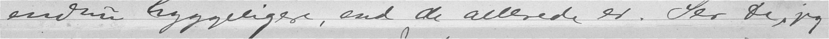endnu hyggeligere, end de allerede er. Ser de, jeg
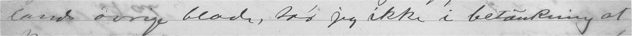lands øvrige blad, tar jeg ikke i betraktning at
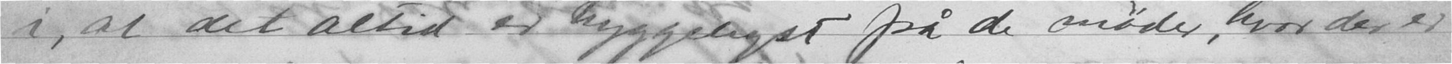i, at det alltid er hyggeligst på de møder, hvor der er
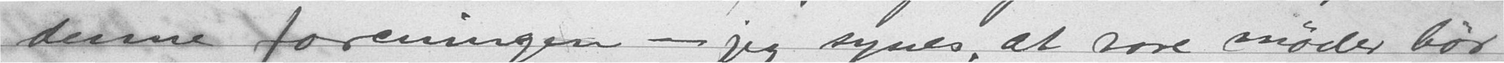denne foreningen - jeg synes, at vore møder bør
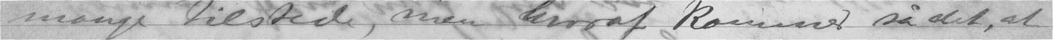mange tilstede, men hvorad kommer så det, at
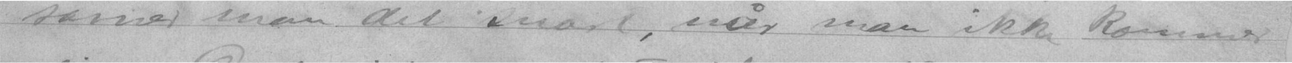savner man det snart, når man ikke kommer
